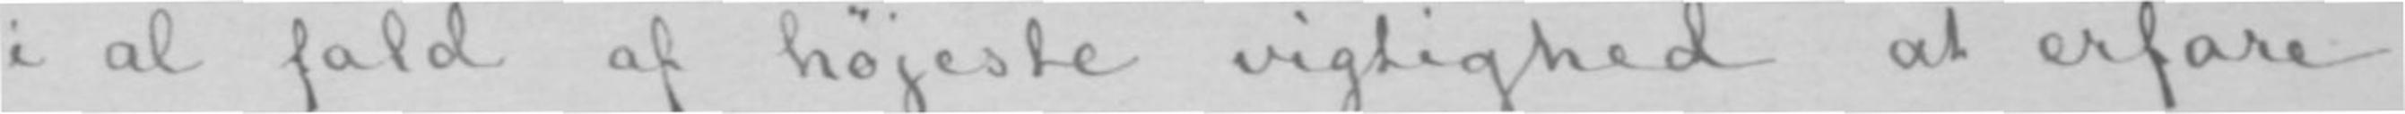i al fald af höjeste vigtighed at erfare
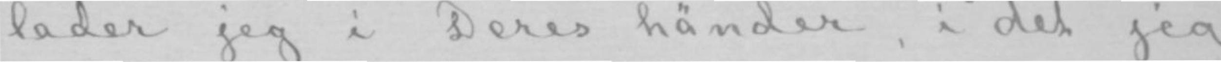lader jeg i Deres händer, i det jeg
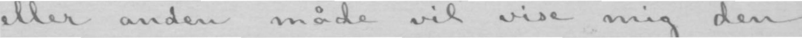eller anden måde vil vise mig den
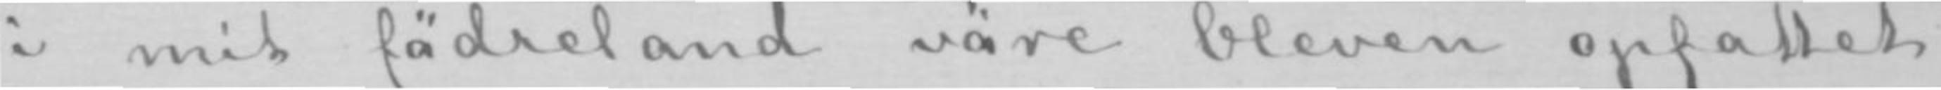i mit fädreland väre bleven opfattet
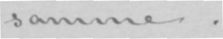samme.
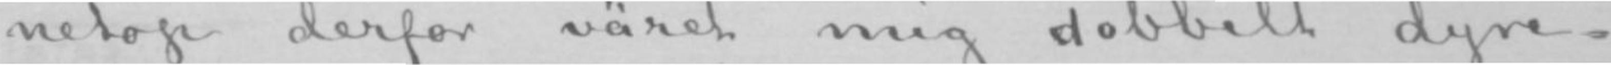netop derfor väret mig dobbelt dyre-
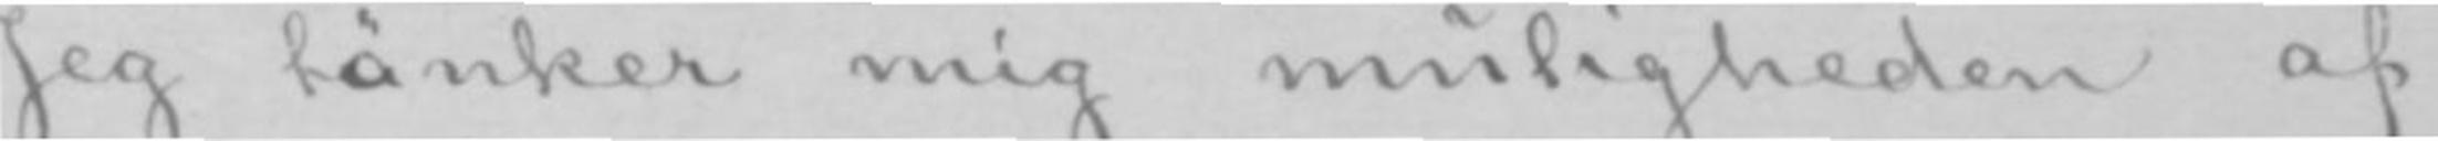Jeg tänker mig muligheden af
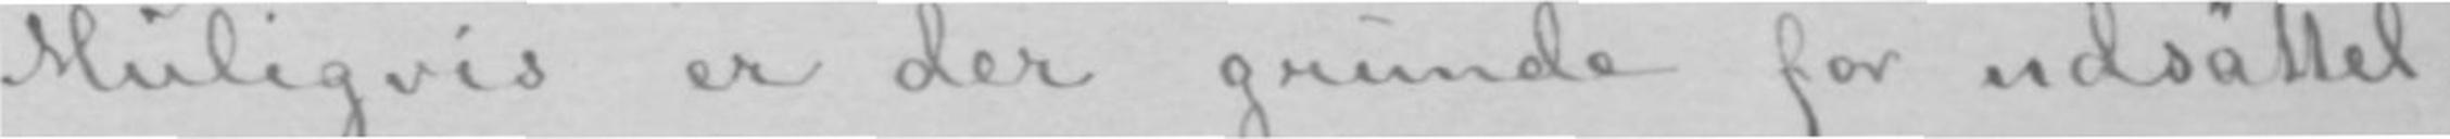Muligvis er der grunde for udsättel-
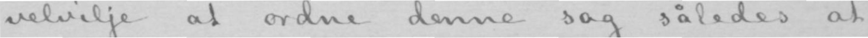velvilje at ordne denne sag således at
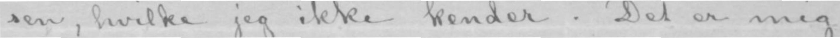sen, hvilke jeg ikke kender. Det er mig
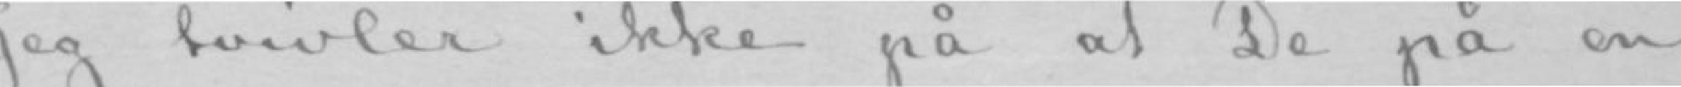Jeg tvivler ikke på at De på en
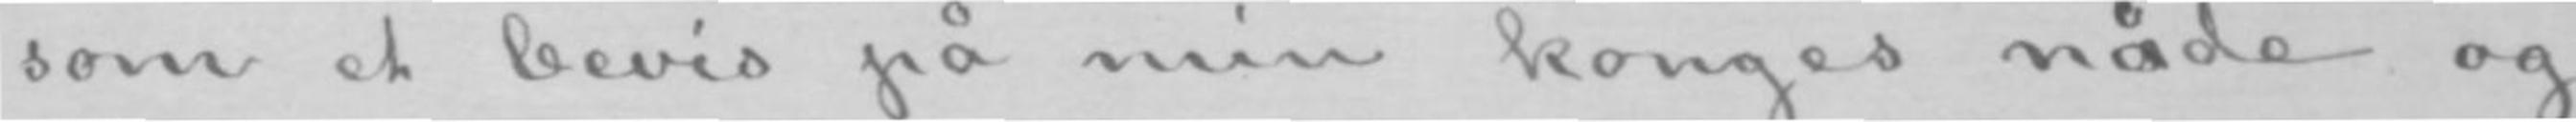som et bevis på min konges nåde og
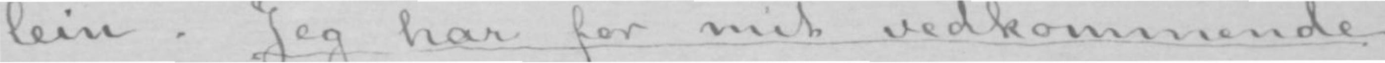lein. Jeg har for mit vedkommende
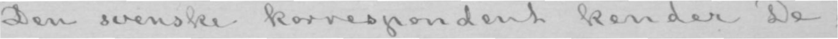Den svenske korrespondent kender De;
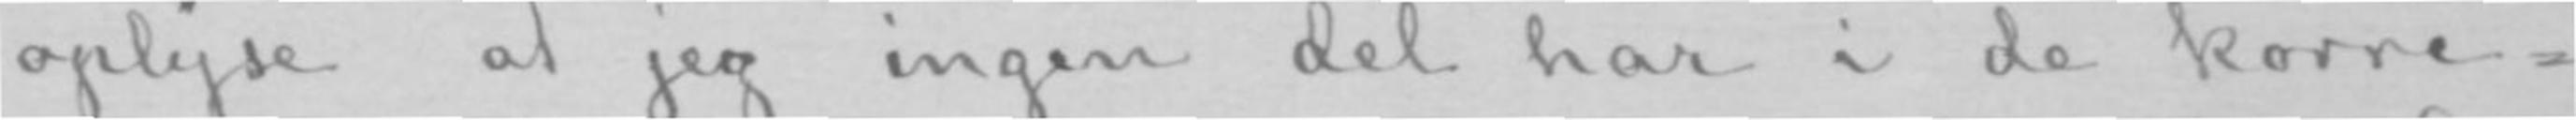oplyse at jeg ingen del har i de korre-
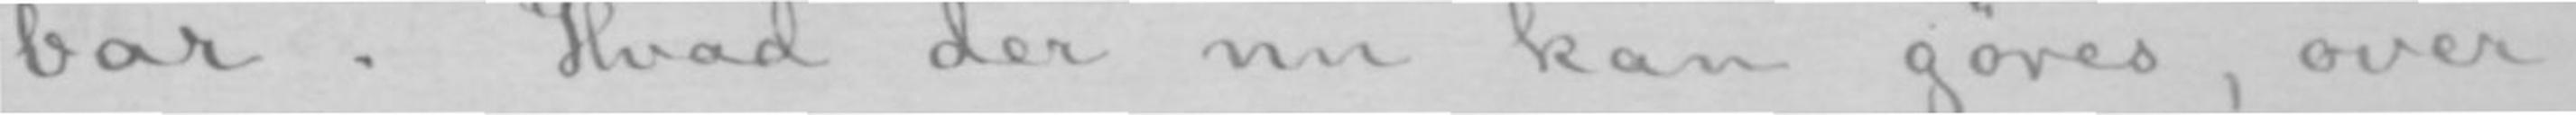bar. Hvad der nu kan göres, over-
